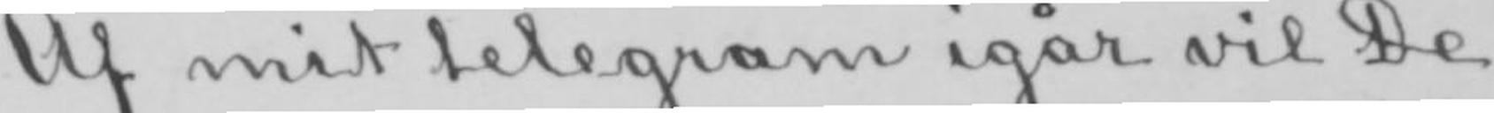Af mit telegram igår vil De
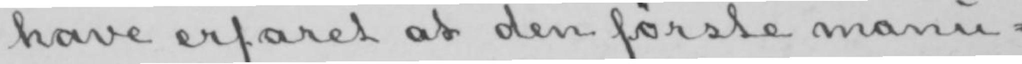have erfaret at den første manu-
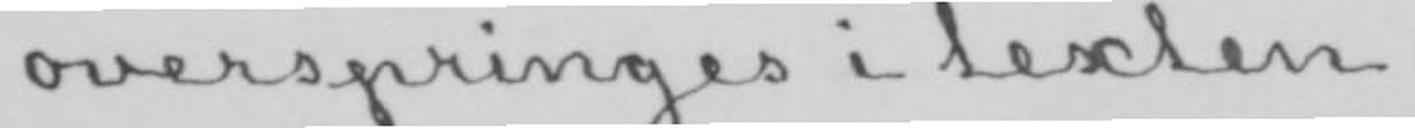overspringes i texten.
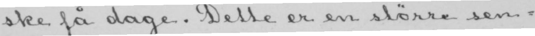ske få dage. Dette er en større sen-
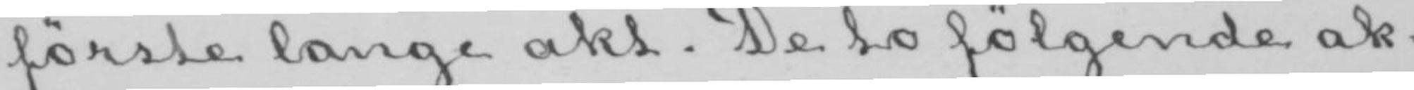første lange akte. De to følgende ak-
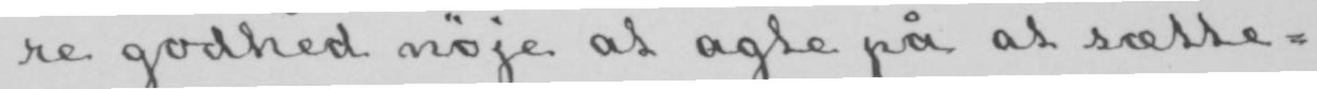re godhed nøje at agte på at sætte-
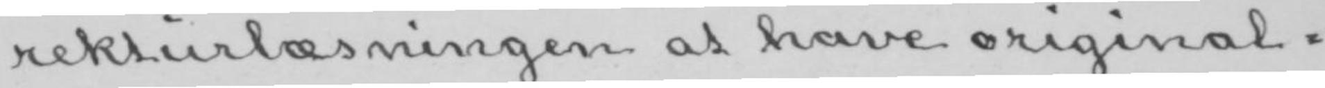rekturlæsningen at have original-
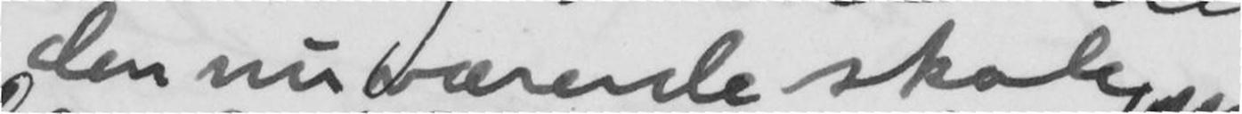den nuværende skole,
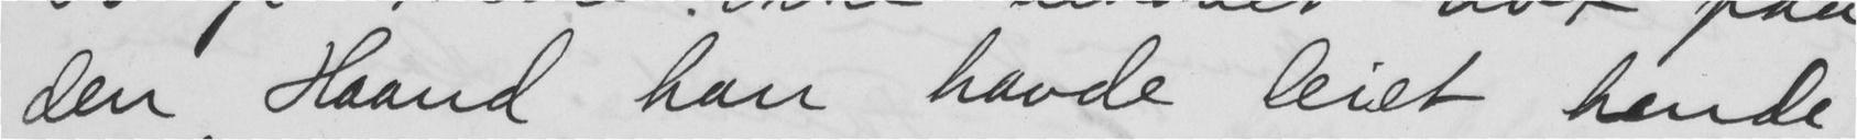den Haand han havde leiet hende
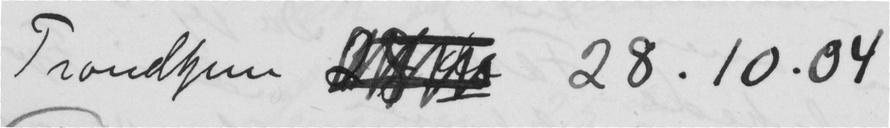Trondhjem  28.10.04
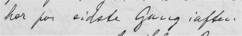her for sidste Gang iaften.
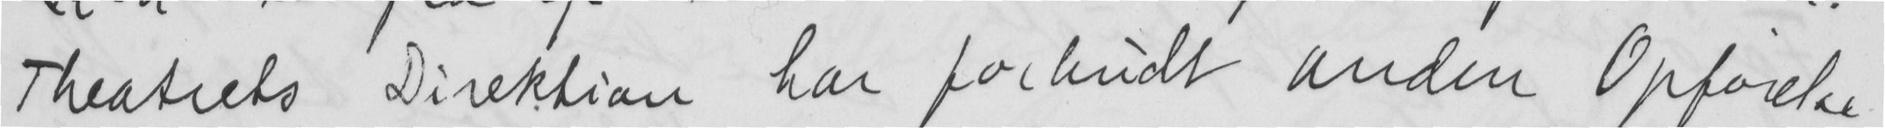Theatrets Direktion har forbudt anden Optørelse
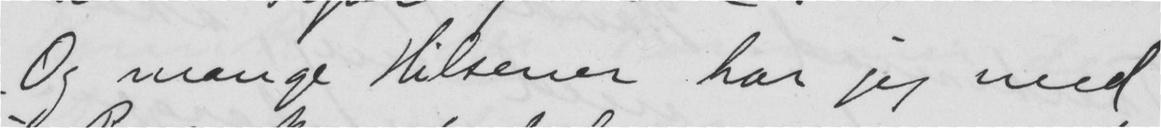Og mange Hilsener har jeg med
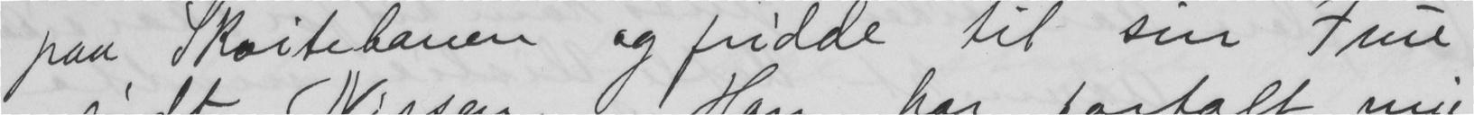paa Skøitebanen og fridde til sin Frue,
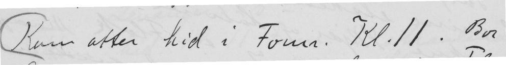Kom atter hid i Form. Kl. 11. Bor
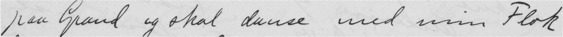paa Grand og skal danse med min Flok
